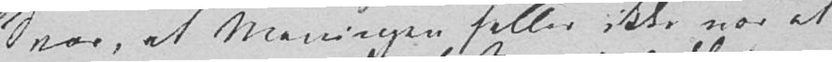Svar, at Meningen heller ikke var at
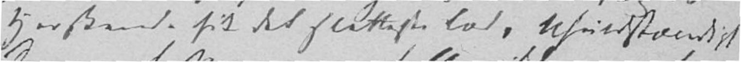Herskende fik det sletteste Lod, Ufuldstændigt
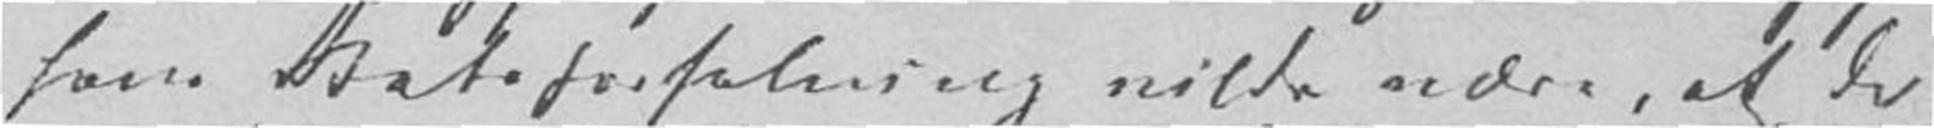hans Statsforfatning vilde være, at de
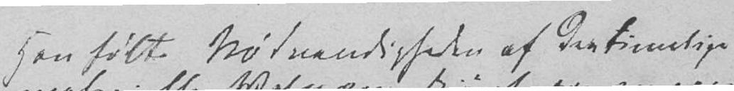Han følte Nødvendigheden af den timelige
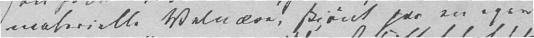materielle Velvære, skjønt paa en egen
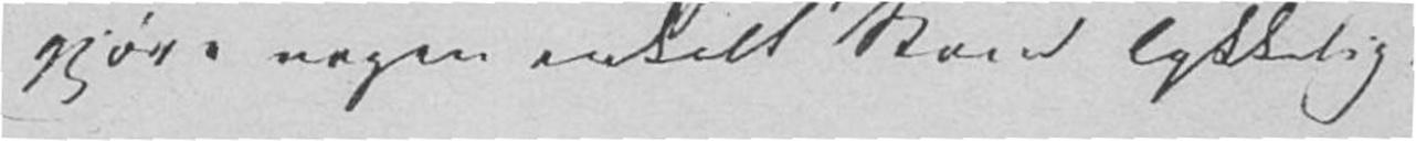gjøre nogen enkelt Stand lykkelig.
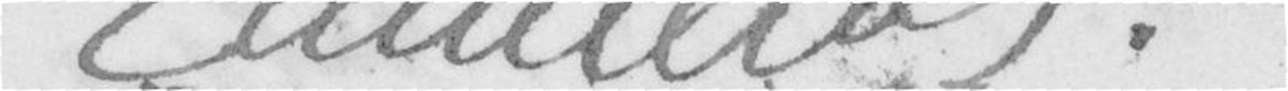Camilla C.
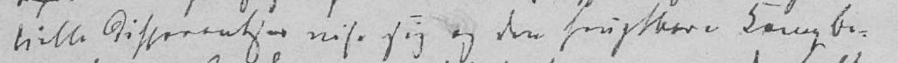pielle Differentser vise sig og den frugtbare Kamp be-
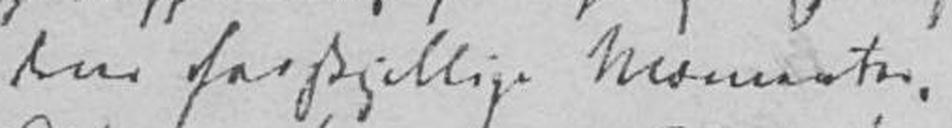dens forskjellige Momenter,
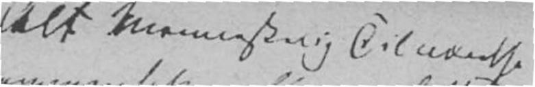Alt menneskelig Tilværelse
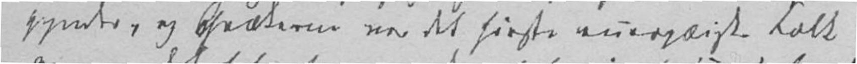gynder, og Grækerne var det første europæiske Folk
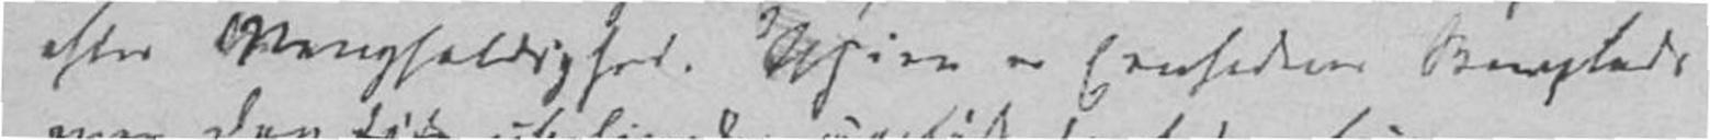efter Mangfoldighed. I Asien er Eenhedens Skueplads
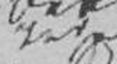indre
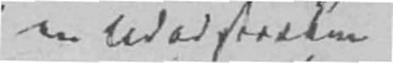en Udadstræben
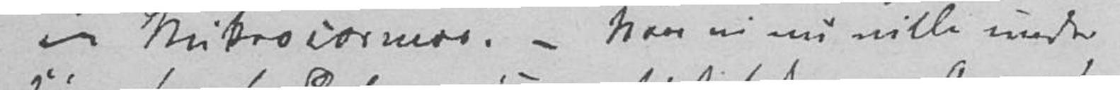– Mikrocosmos. - Naar vi nu ville under-
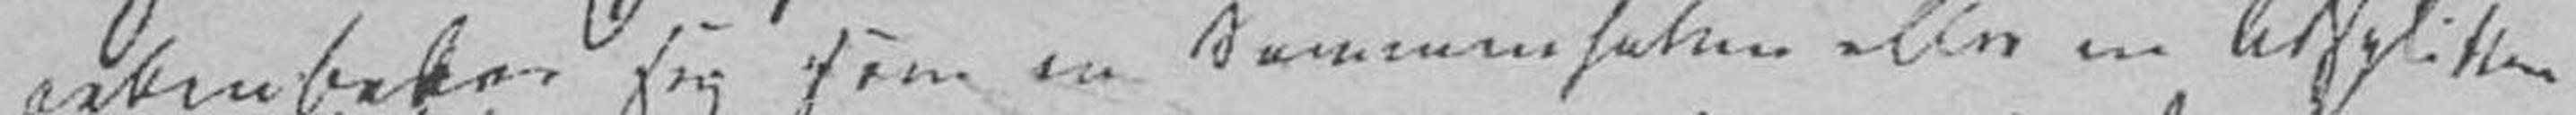aabenbarer sig som en Sammenfatten eller en Adsplitten
